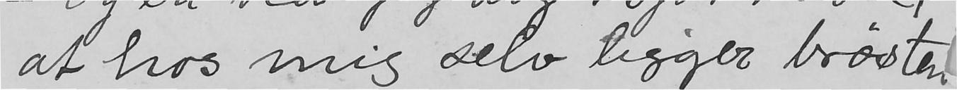at hos mig selv ligger brøsten
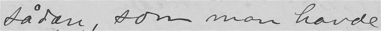sådan, som man havde
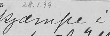kjæmpe i
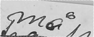må
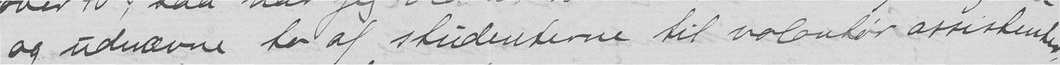og udnævne to af studenterne til volontør assistenter,
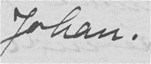Johan.
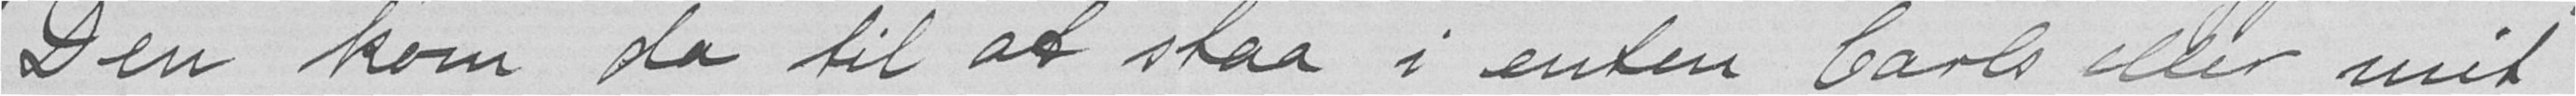Den kom da til at staa i enten Carls eller mit
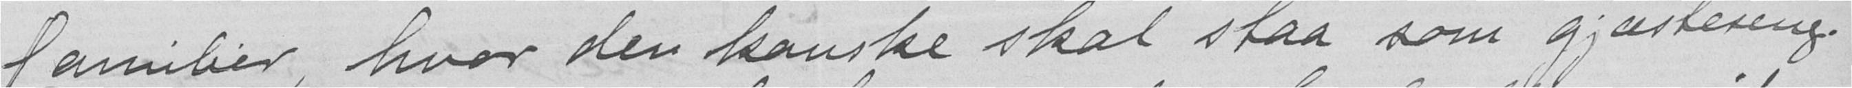familier, hvor den kanske skal staa som gjæsteseng.
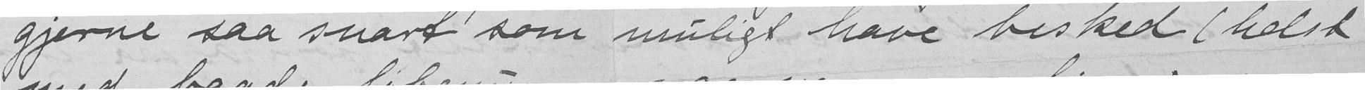gjerne saa snart som muligt have besked (helst
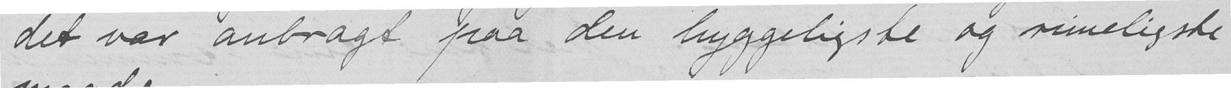det var anbragt paa den hyggeligste og rimeligste
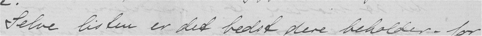Selve listen er det bedst dere beholder - for
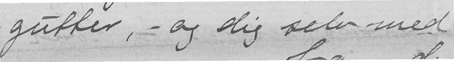gutter, - og dig selv med
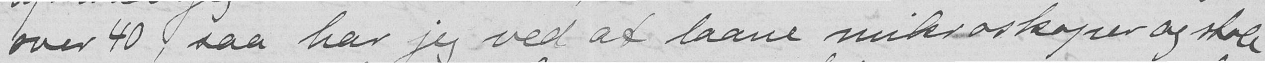over 40, saa har jeg ved at laane mikroskoper og stole
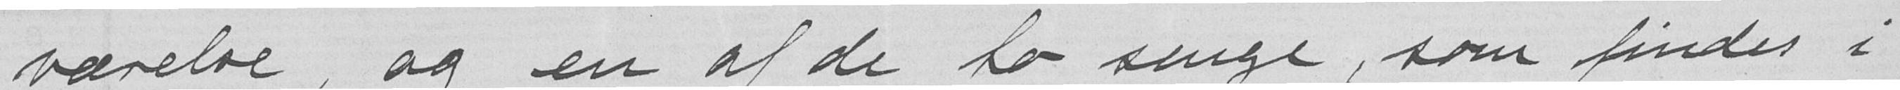værelse, og en af de to senge, som findes i
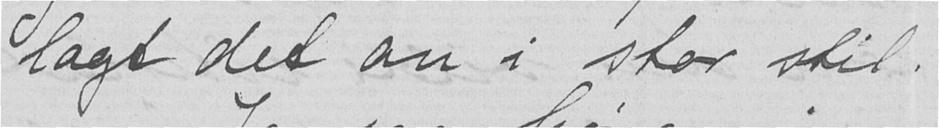lagt det an i stor stil.
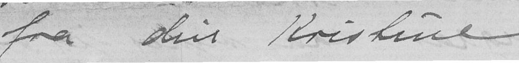fra din Kristine.
